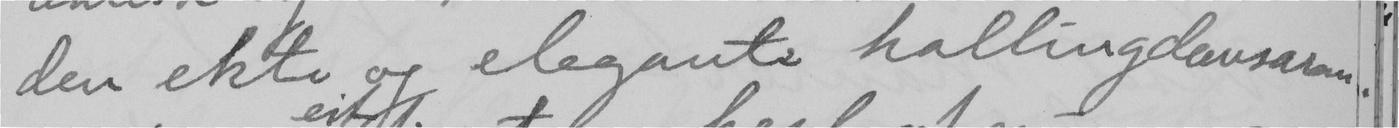den ekte og elegante hallingdansaren.
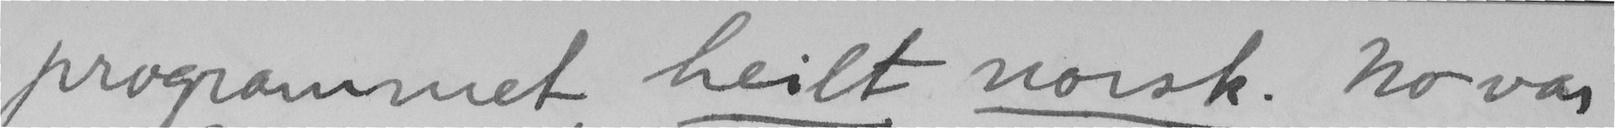programmet heilt norsk. No var
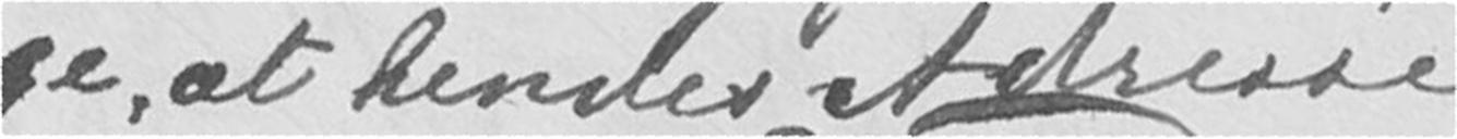ge, at hendes Adresse
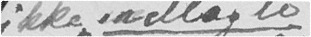ikke "indlæg to
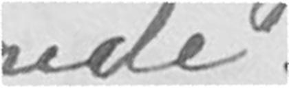nede.
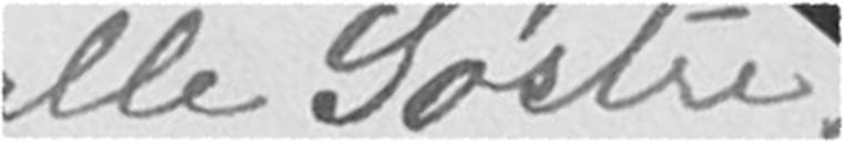alle Søstre-
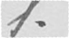1
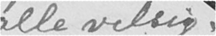alle velsig-
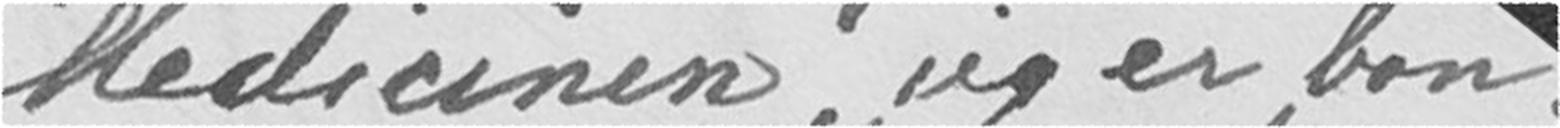Medicinen, jeg er ban-
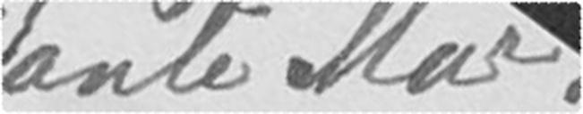Tante Mar-
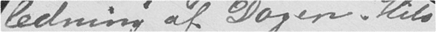ledning af Dagen. Hils
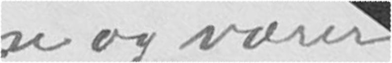ne og varer
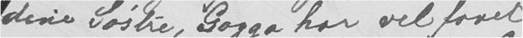dine Søstre, Gagga har vel faaet
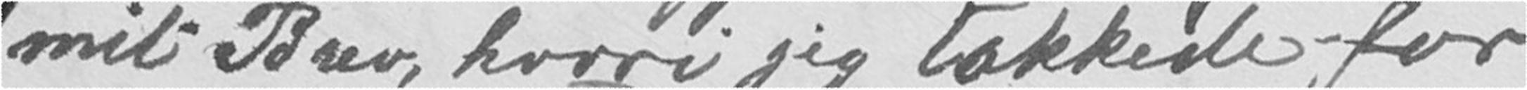mit Brev, hvori jeg takket for
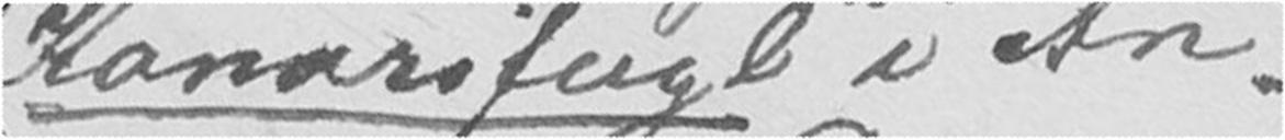Kanarifugl" i An-
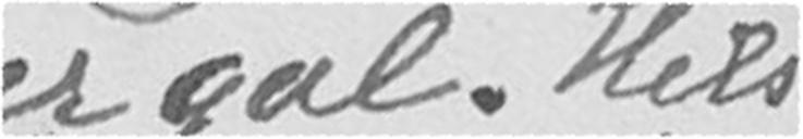er gal. Hils
